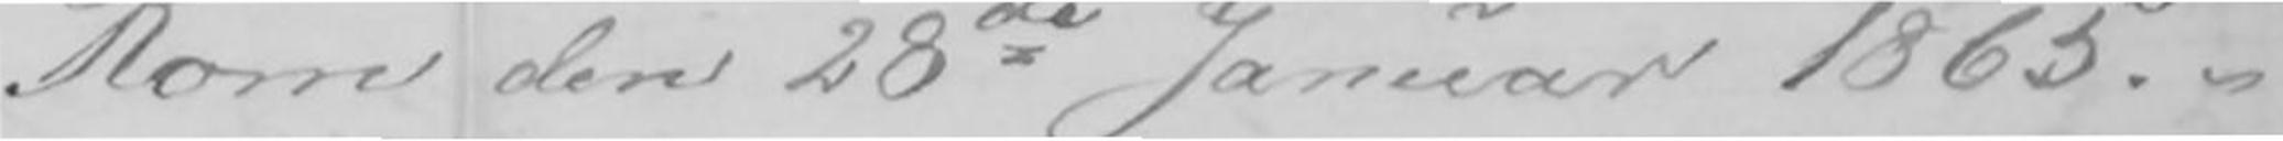Rom den 28de Januar 1865.-
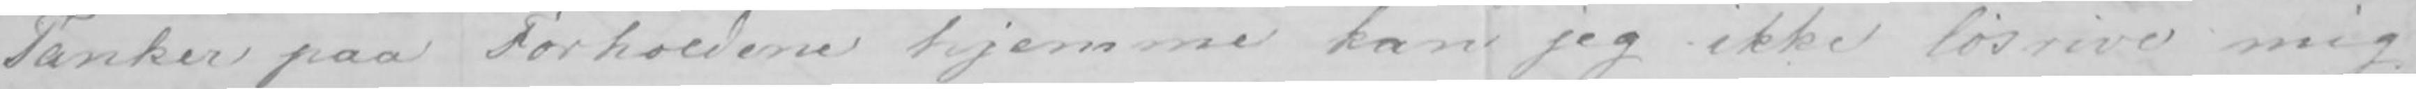Tanker paa Forholdene hjemme kan jeg ikke løsrive mig
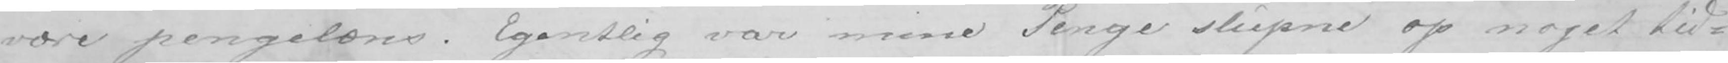være pengelæns. Egentlig var mine Penge slupne op noget tid-
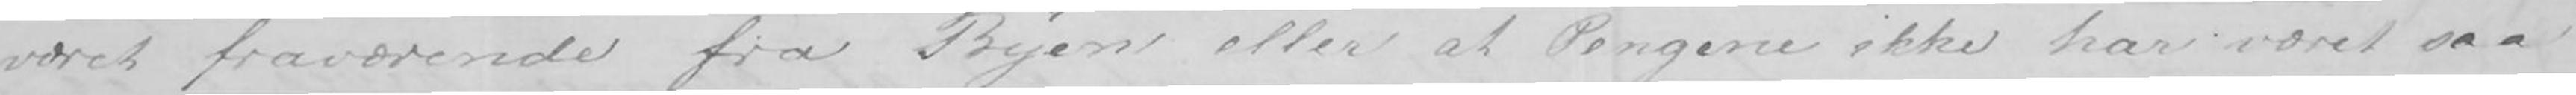været fraværende fra Byen eller at Pengene ikke har været saa
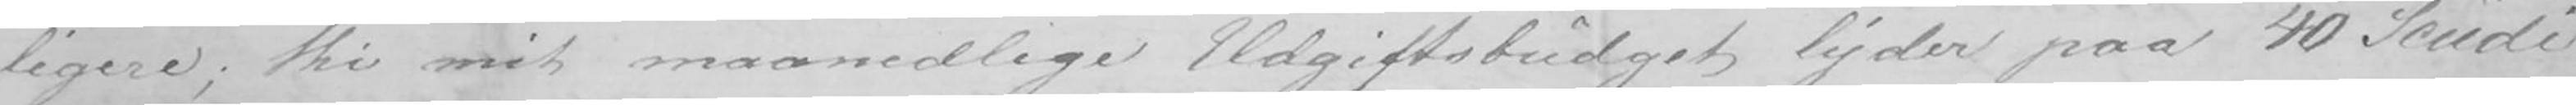ligere; thi mit maanedlige Udgiftsbudget lyder paa 40 Scudi
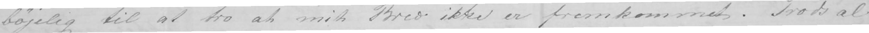bøjelig til at tro at mit Brev ikke er fremkommet. Trods al
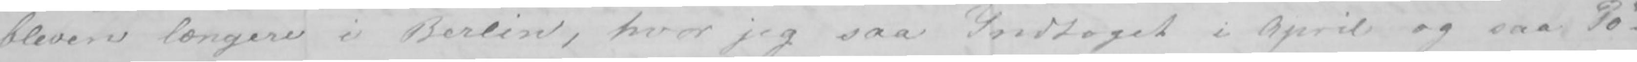bleven længere i Berlin, hvor jeg saa Indtoget i April og saa Pø-
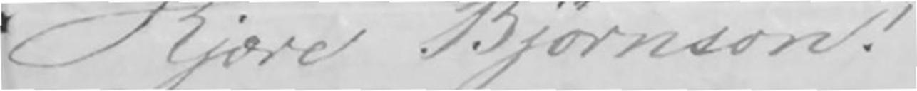Kjære Bjørnson!
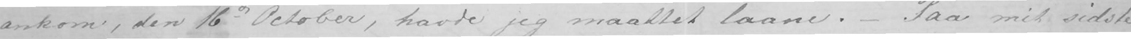ankom, den 16de October, havde jeg maattet laane.- Paa mit sidste
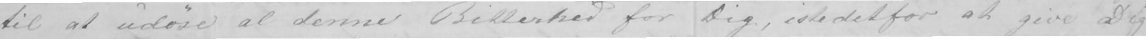til at udøse al denne Bitterhed for Dig, istedetfor at give Dig
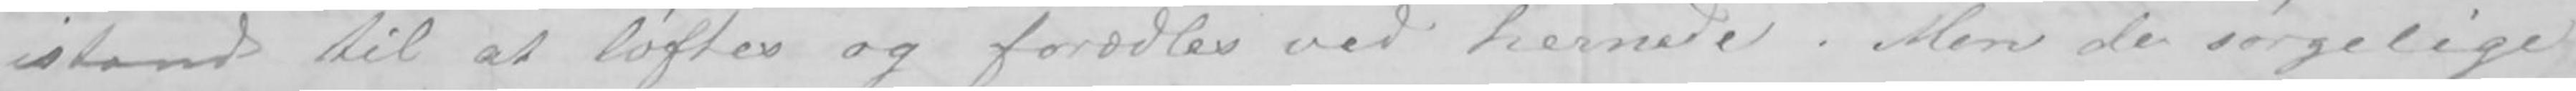istand til at løftes og forædles ved hernede. Men de sørgelige
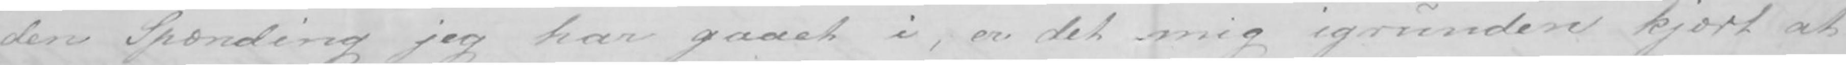den Spænding jeg har gaaet i, er det mig igrunden kjært at
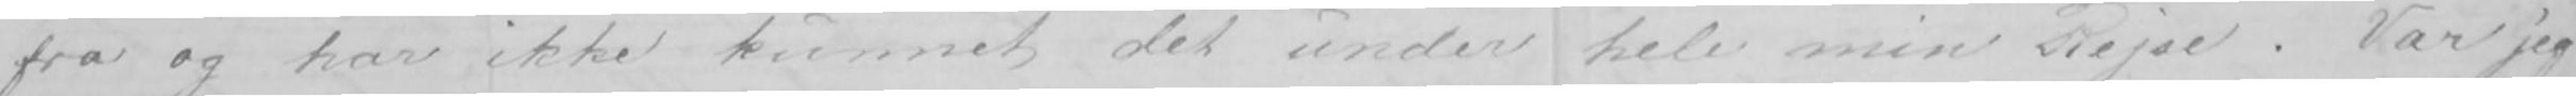fra og har ikke kunnet det under hele min Rejse. Var jeg
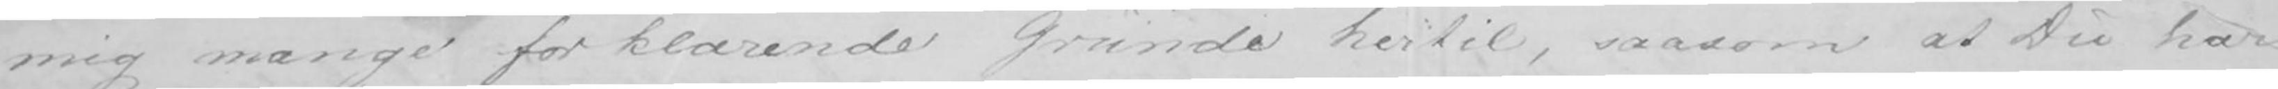mig mange forklarende Grunde hertil, saasom at Du har
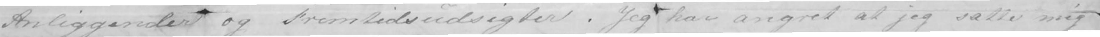Anliggender og Fremtidsudsigter. Jeg har angret at jeg satte mig
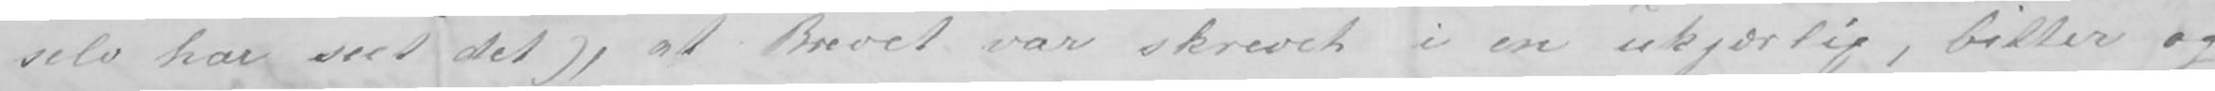selv har seet det), at Brevet var skrevet i en ukjærlig, bitter og
